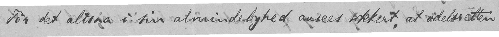Før det altsaa i sin almindelighed ansees sikkert, at odelsretten
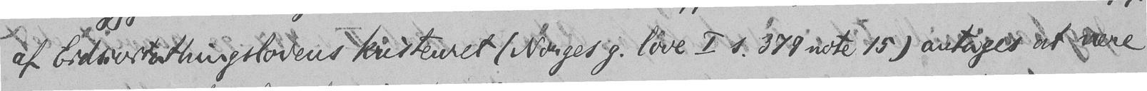af Eidsivathingslovens kristenret (Norges g. love I s. 379 note 15) antages at være
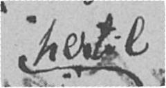hertil
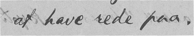at have rede paa.
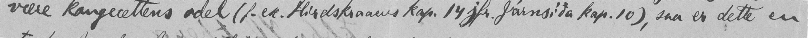være kongeættens odel (f. ex. Hirdskraaens kap. 14 jfr. Járnsíða kap. 10), saa er dette en
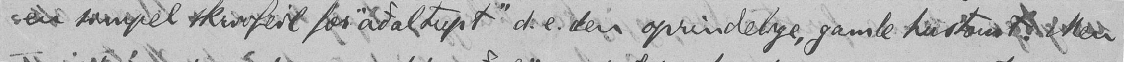en simpel skrivefeil for "oðalstupt" d. e. den oprindelige, gamle hustomt. Men
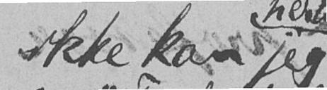ikke kan jeg
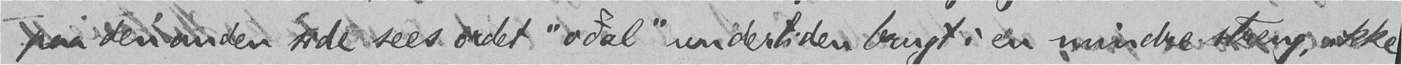paa den anden side sees ordet "oðal" undertiden brugt i en mindre streng, ikke
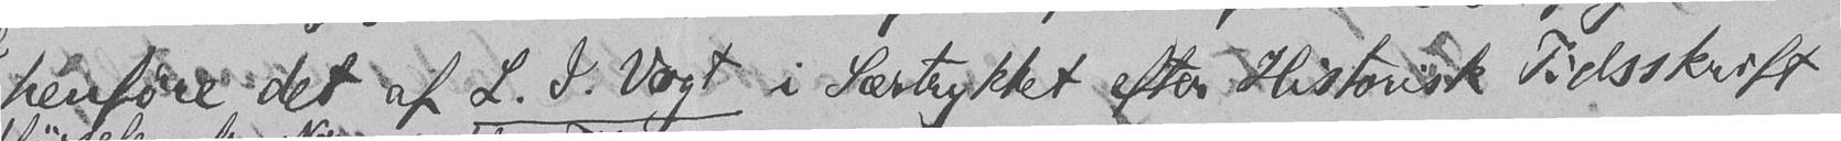henføre det af L. J. Vogt i Særtrykket efter Historisk Tidsskrift
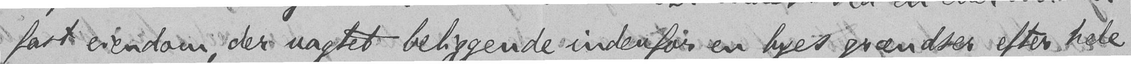fast eiendom, der uagtet beliggende indenfor en byes grændser efter hele
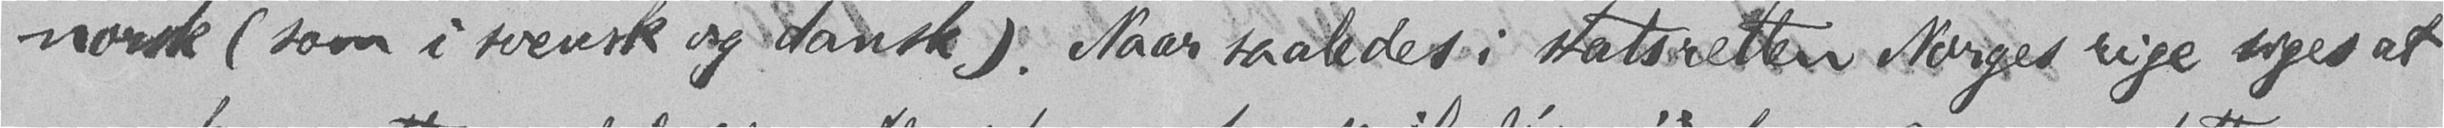norsk (som i svensk og dansk). Naar saaledes i statsretten Norges rige siges at
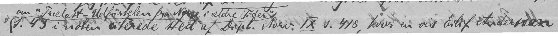s. 43 i noten citerede sted af Dipl. Norv. IX s. 418, hvor en vis Eilif Andersson
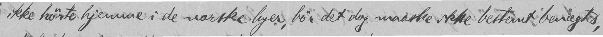ikke hørte hjemme i de norske byer, bør det dog maaske ikke bestemt benægtes,
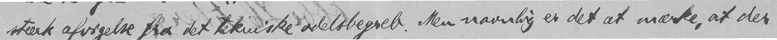stærk afvigelse fra det tekniske odelsbegreb. Men navnlig er det at mærke, at der
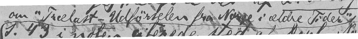om "Trælast-Udførselen fra Norge i ældre Tider"
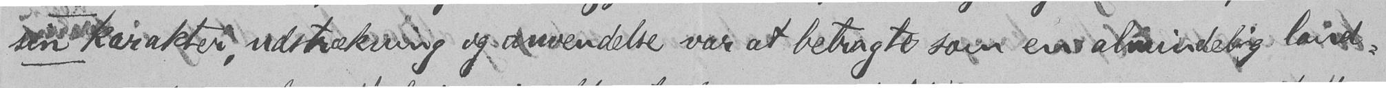sin karakter, udstrækning og anvendelse var at betragte som en almindelig land-
